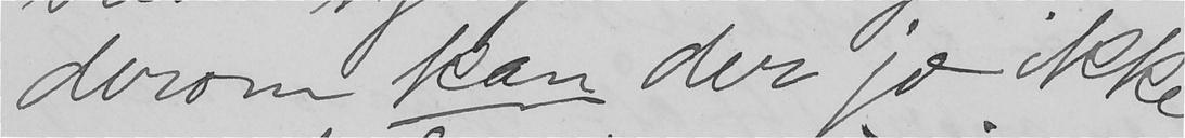derom kan der jo ikke
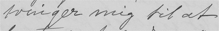tvinger mig til at
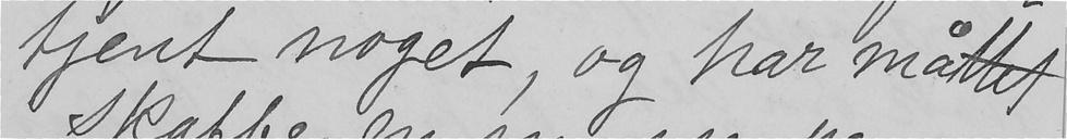tjent noget, og har måttet
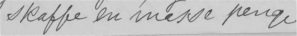skaffe en masse penge
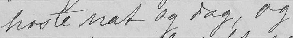hoste nat og dag, og
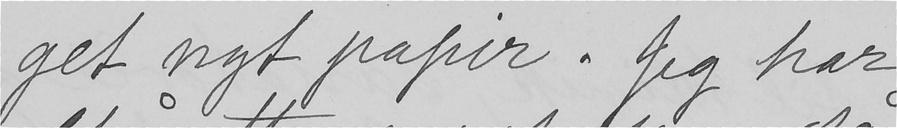get nyt papir. Jeg har
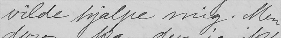vilde hjælpe mig. Men
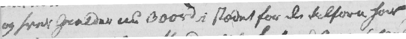og hver gielder nu 300 rd i Stædet for de tilforn har
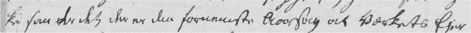ke som der det der er den fornemste Aarsag at Værkets Ejere
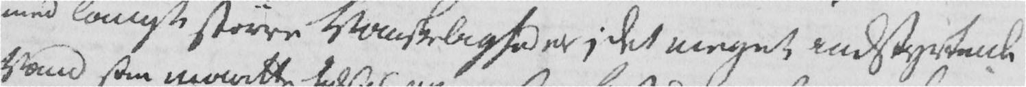med langt større Vanskeligheder; det meget indstyrtende
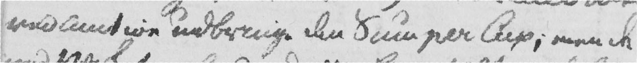ved Auction udbringe den Sum per Cux; men de
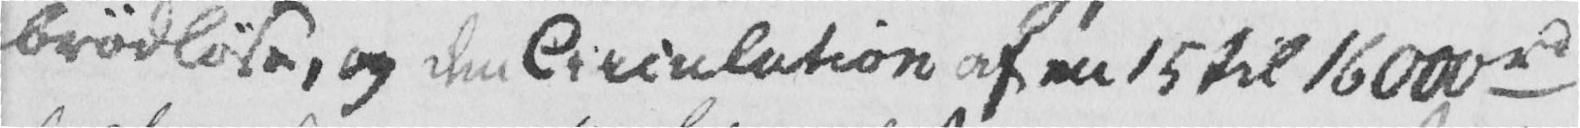brødløse, og den Circulation af en 15 til 16000 rd
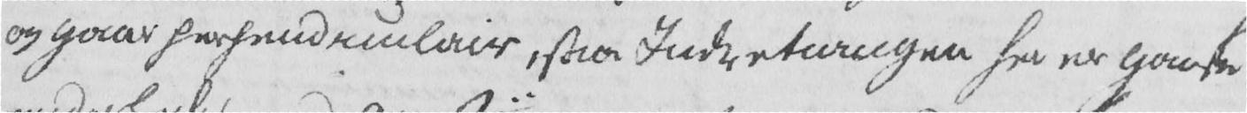og gaar perpendiculair, saa Indretningen her er ganske
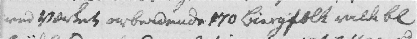ved Værket arbeidende 170 Biergfolk vilde bl
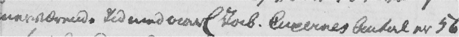nerværende Tid med aarl Tab. Cuxernes Antal er 56
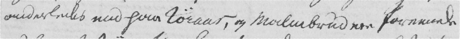anderledes end paa Røraas, og Malmbrudene forenede
